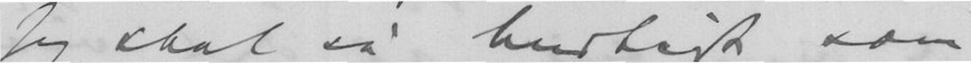Jeg skal så hurtigt som
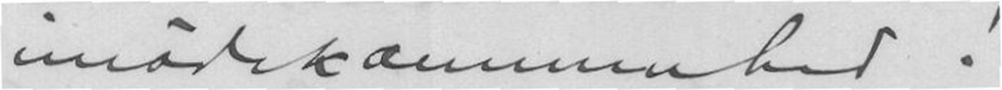imødekommenhed!
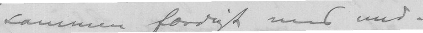sammen færdigt med med-
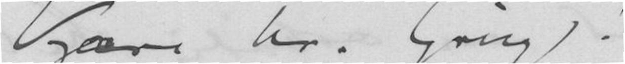Kjære hr. Grieg!
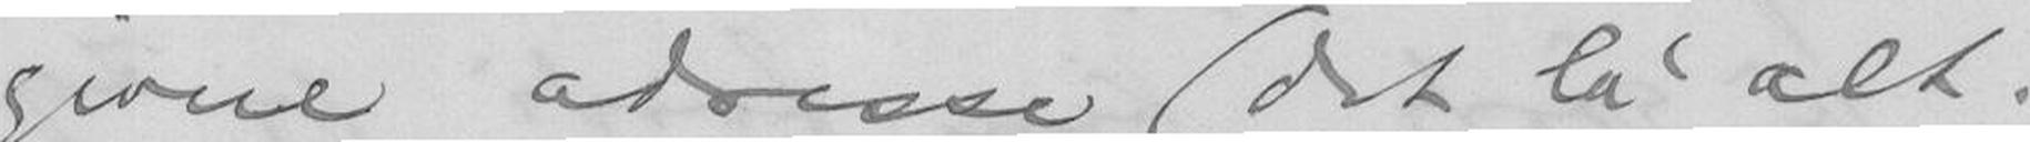givne adresse (det lå alt
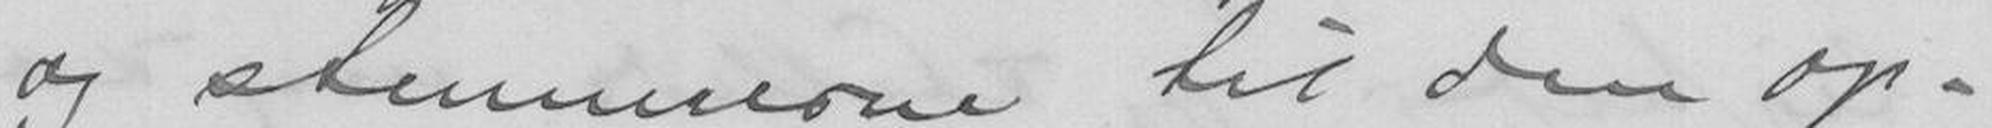og stemmerne til den op-
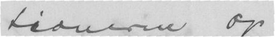tionerne op.
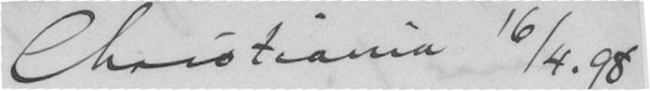Christiania 16/4.98
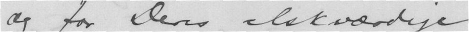og for Deres elskværdige
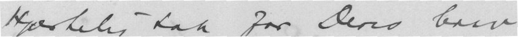Hjærtelig tak for Deres brev
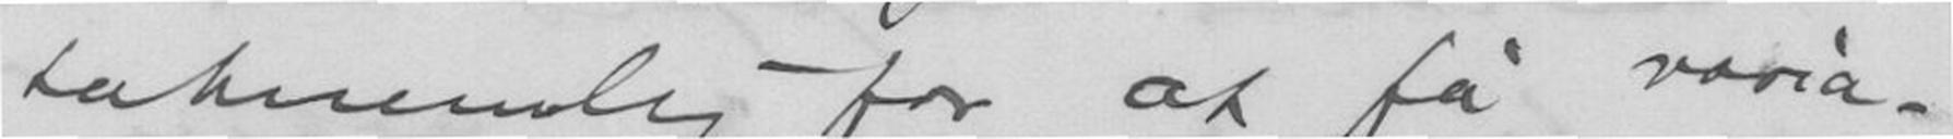taknemlig for at få varia-
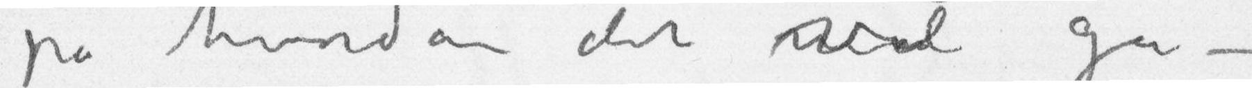på hvordan det vil gå –
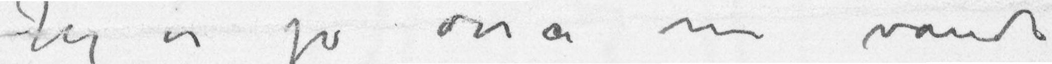Jeg er jo osså nu vandt
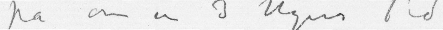på om en 3 Ugers Tid
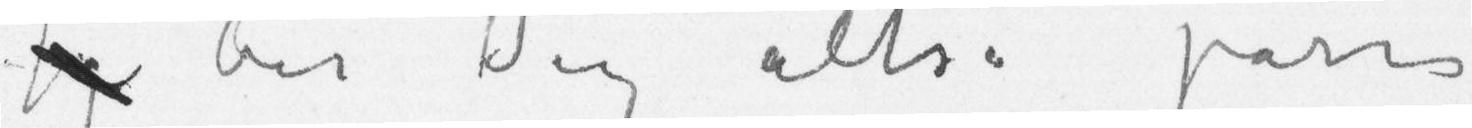Jeg ber Dig altså passe
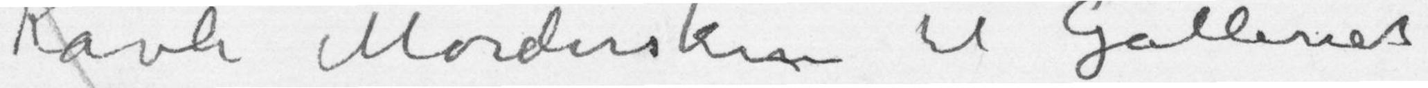Kavli Mordersken til Galleriet
